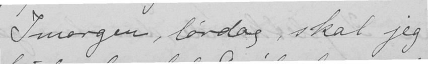Imorgen, lørdag, skal jeg
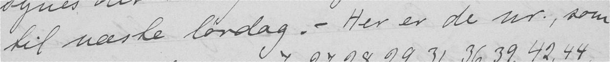til næste lørdag. - Her er de nr., som
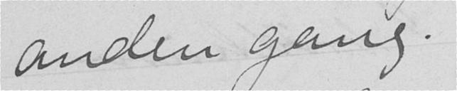anden gang.
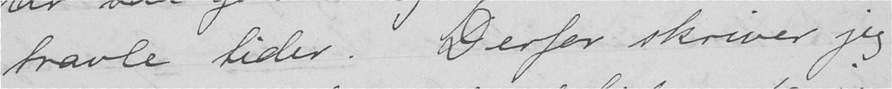travle tider. Derfor skriver jeg
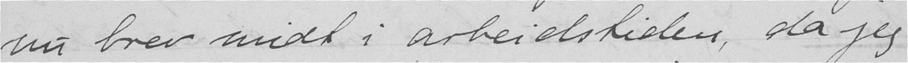nu brev midt i arbeidstiden, da jeg
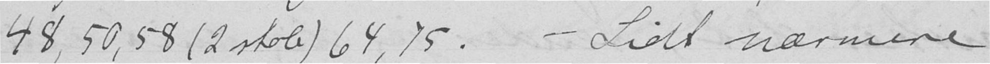48, 50, 58 (2 stole) 64, 75. - Lidt nærmere
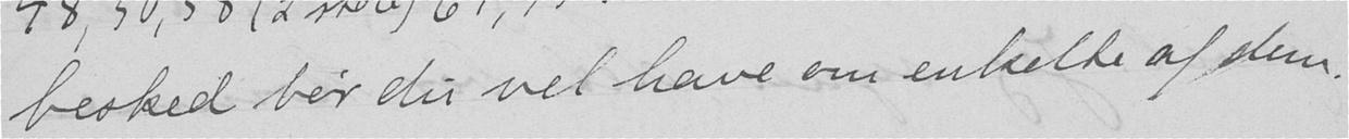besked bør du vel have om enkelte af dem.
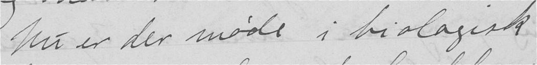Nu er der møde i biologisk
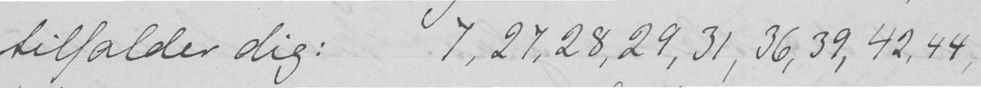tilfalder dig: 7, 27, 28, 29, 31, 36, 39, 42, 44,
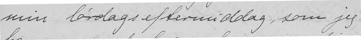min lørdagseftermiddag, som jeg
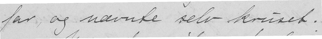far, og nævnte selv kruset.
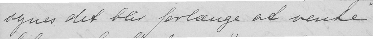synes det blir forlænge at vente
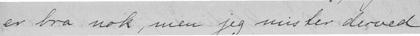er bra nok, men jeg mister derved
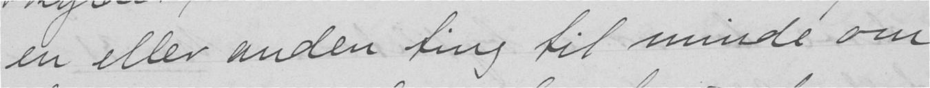en eller anden ting til minde om
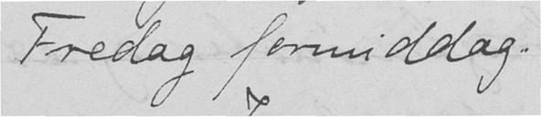Fredag formiddag.
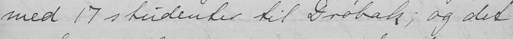med 17 studenter til Drøbak; og det
